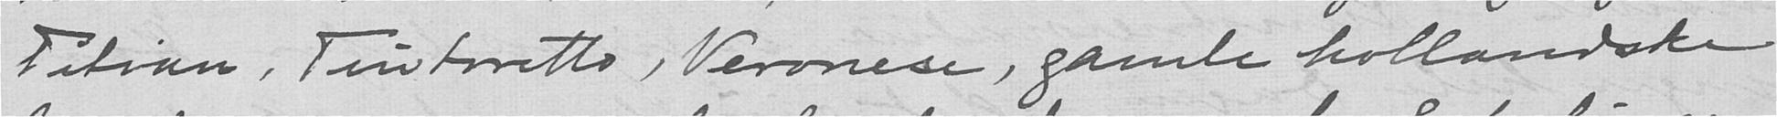Titian, Tintoretto, Veronese, gamle hollandske
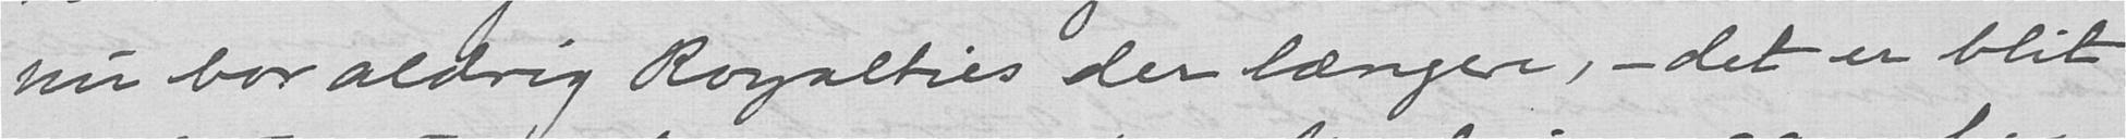nu bor aldrig Royalties der længere, - det er blit
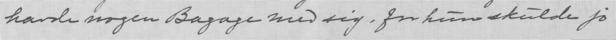havde nogen Bagage med sig, for hun skulde jo
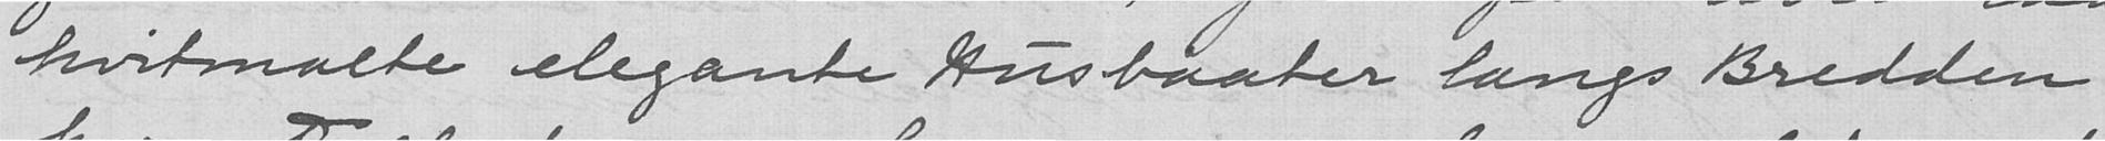hvitmalte elegante Husbaater langs Bredden,
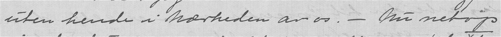uten hende i nærheden av os. - Nu netop
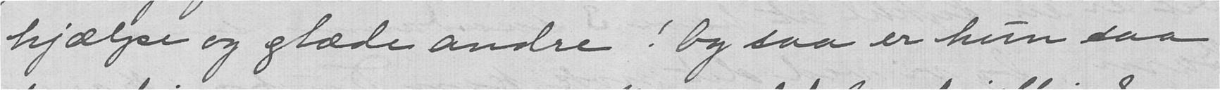hjælpe og glæde andre! Og saa er hun saa
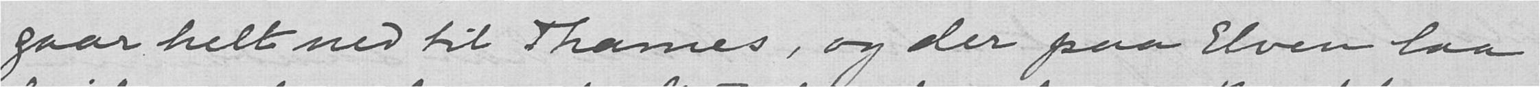gaar helt ned til Thames, og der paa Elven laa
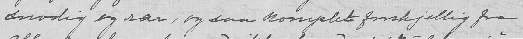snodig og rar, og saa komplet forskjellig fra
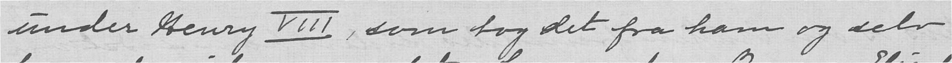under Henry VIII, som tog det fra ham og selv
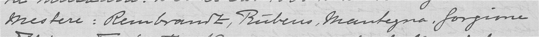Mestere: Rembrandt, Rubens, Mantegna, forgivne,
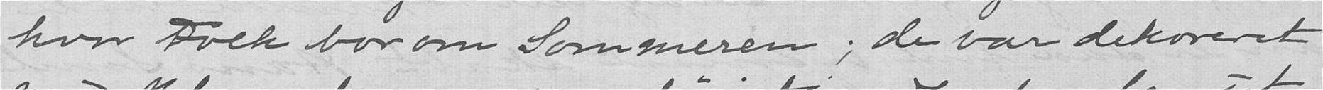hvor Folk bor om Sommeren; de var dekoreret
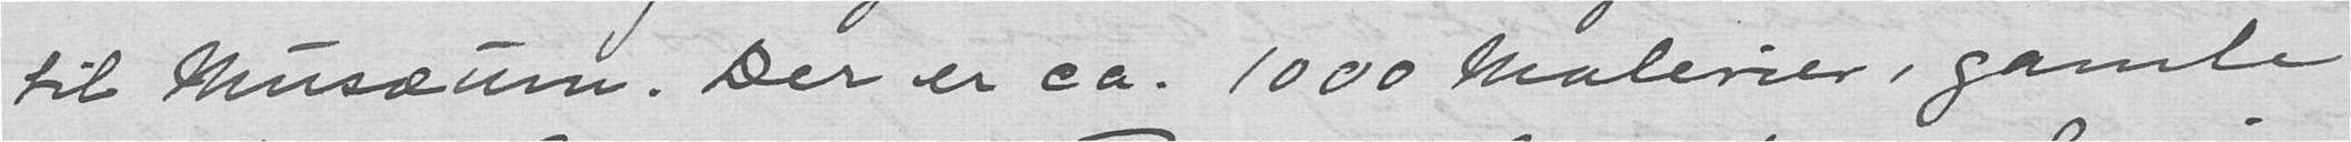til Musæum. Der er ca. 1000 Malerier, gamle
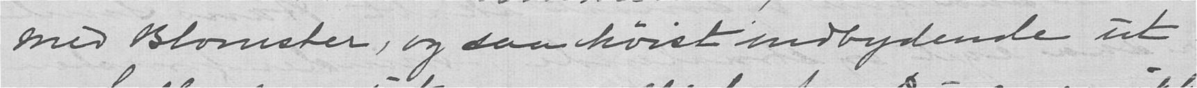med Blomster, og saa høist indbydende ut.
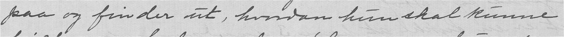paa og finder ut, hvordan hun skal kunne
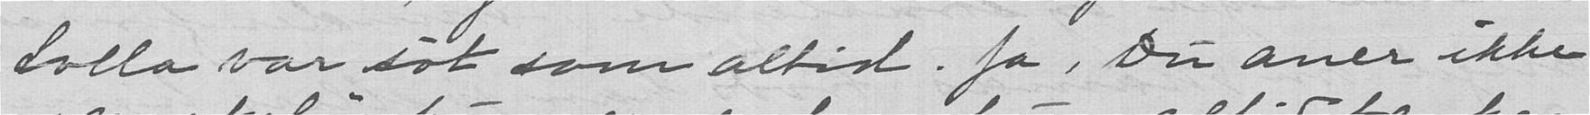Lolla var søt som altid. Ja, Du aner ikke
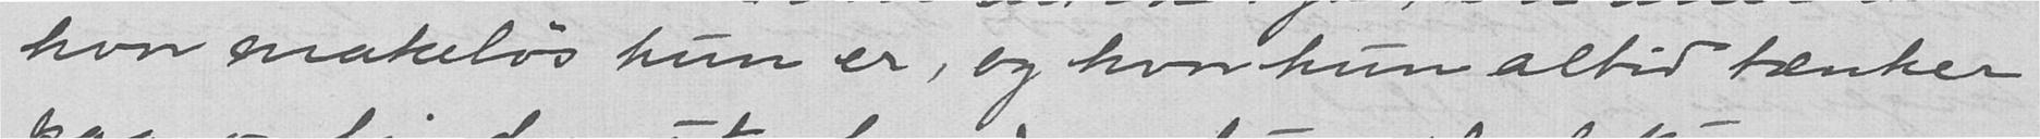hvor makeløs hun er, og hvor hun altid tænker
